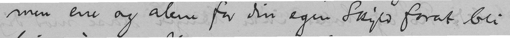men ene og alene for din egen Skyld forat bli
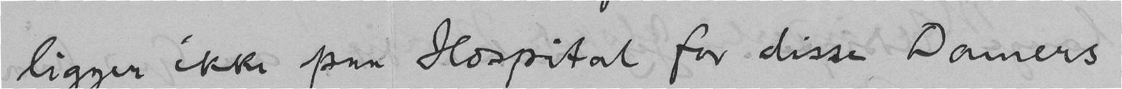ligger ikke paa Hospital for disse Damers
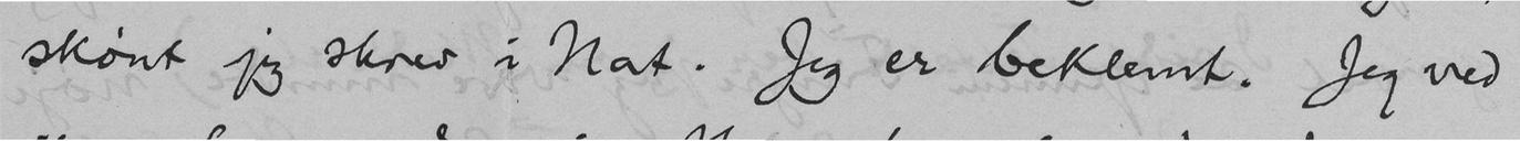skønt jeg skrev i Nat. Jeg er beklemt. Jeg ved
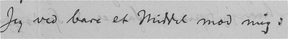Jeg ved bare et Middel mod mig:
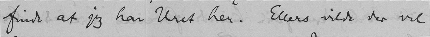finde at jeg har Uret her. Ellers vilde der vel
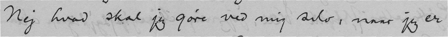Nej hvad skal jeg gøre ved mig selv, naar jeg er
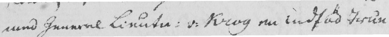med General Lieutn.: v. Krog en indfød Trun-
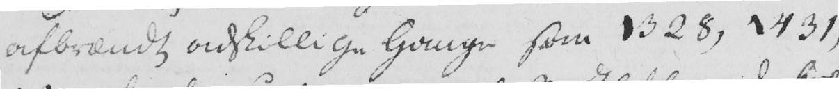afbrændt adskillige Gange som 1328, 1431,
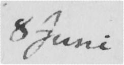8 Juni
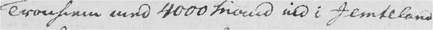Tronhiem med 4000 Mand ind i Jemteland
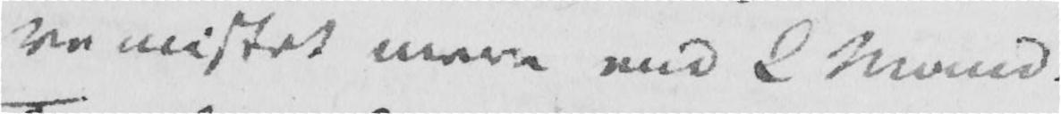ve mistet mere end 2 Mand.
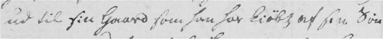ud til sin Gaard som han har kiøbt af sin Søn
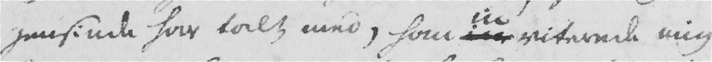gensinde har talt med, som inviterede mig
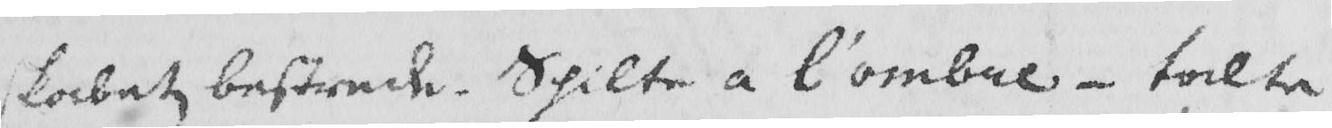skabet bestrede. Spilte a l'ombre - talte
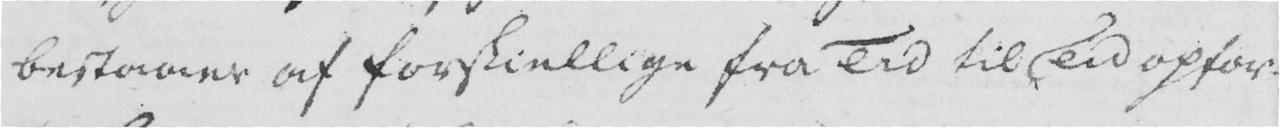bestaaer af forskiellige fra Tid til Tid opfør-
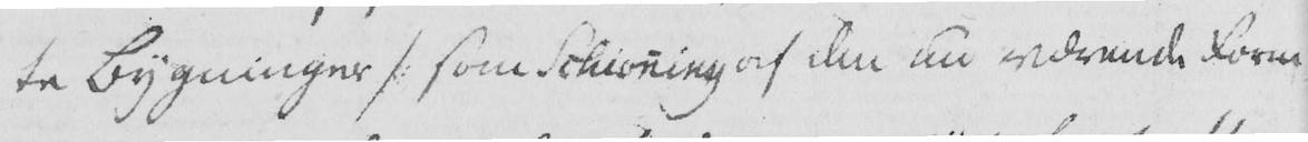te Bygninger /: som Schiøning af den nu værende Form
